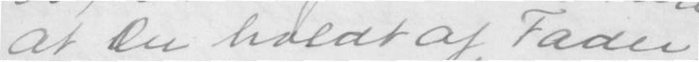at Du holdt af Fader
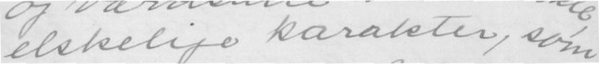elskelige karakter, som
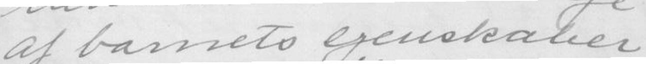af barnets egenskaber
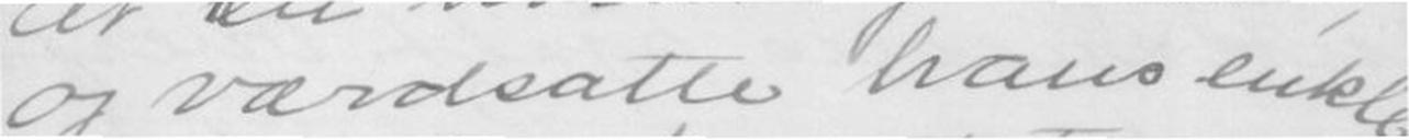og værdsatte hans enkle
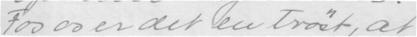For os er det en trøst, at
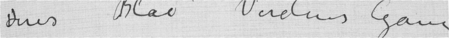Deres Blad Verdens Gang
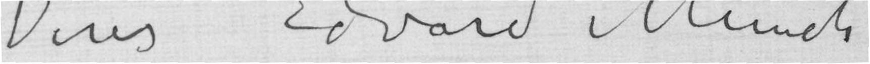Deres Edvard Munch
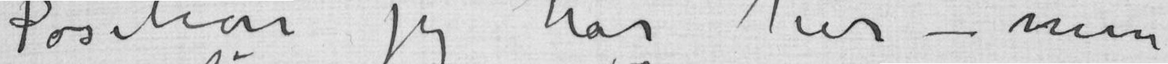Position jeg har her – men
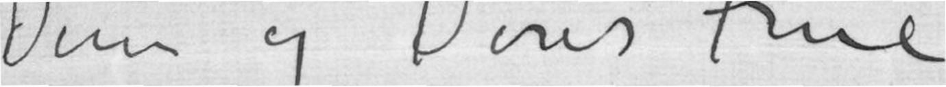Dem og Deres Frue
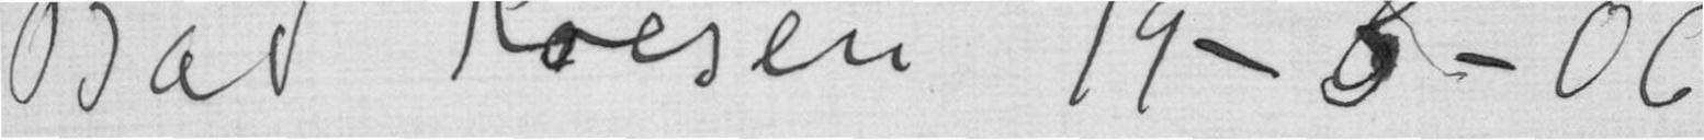Bad Koesen 19-63-06
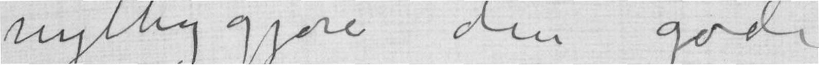nyttiggjøre den gode
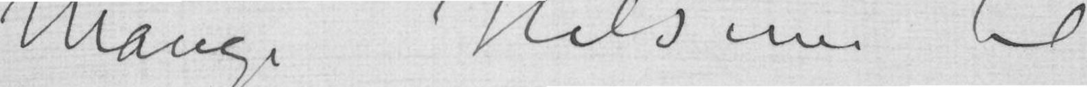Mange Hilsener til
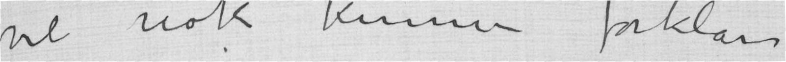vil nok kunne forklare
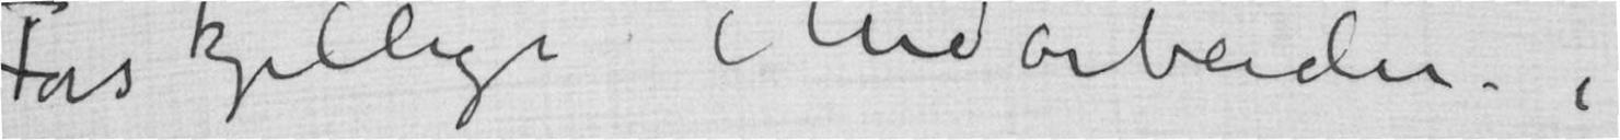Forskjellige Medarbeidere i
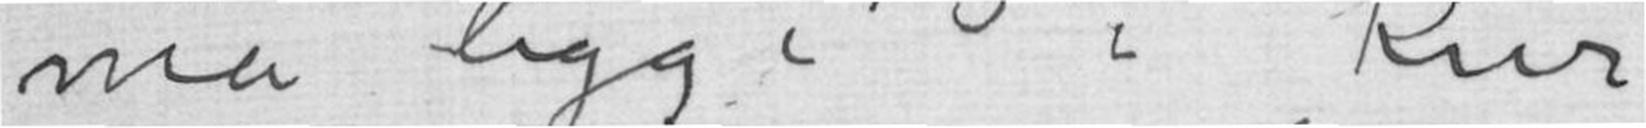må ligge i Kur
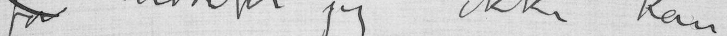for hvorfor jeg ikke kan
